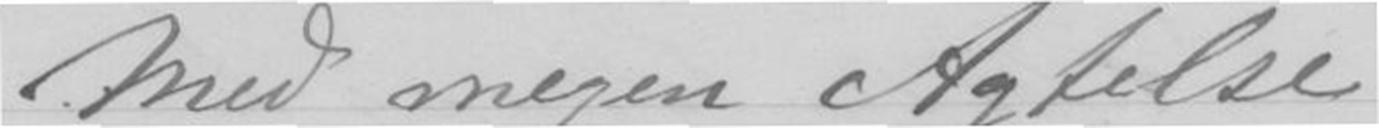Med megen Agtelse
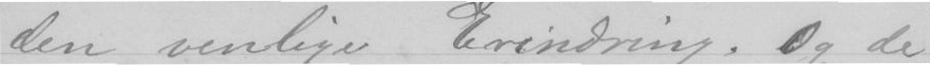den venlige Erindring, og de
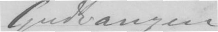Gudvangen
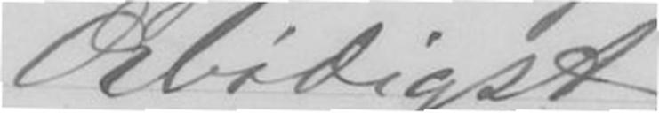Ærbødigst
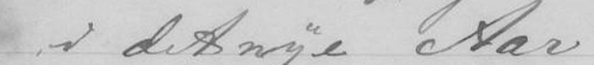i det nye Aar.
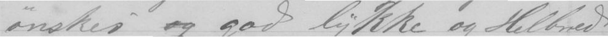ønskes og god lykke og Helbred
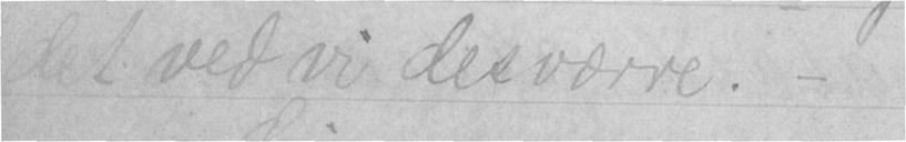det ved vi desværre. -
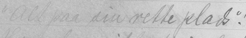"alt paa sin rette plads"!
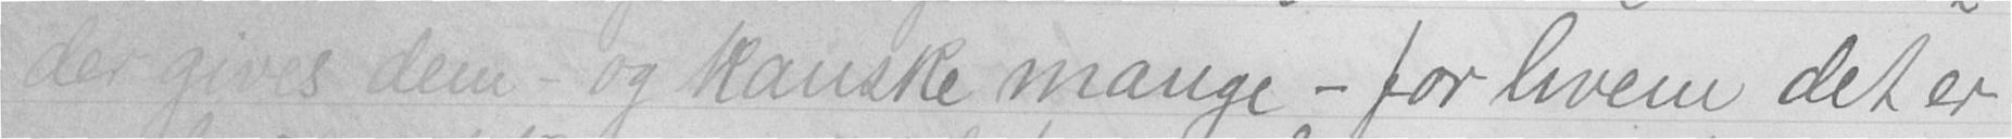der gives dem - og kanske mange - for hvem det er
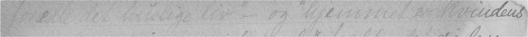fortelle det "huselige liv" - og "hjemmet er kvindens
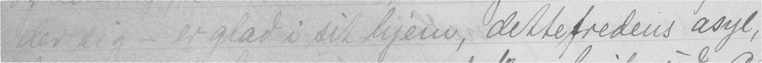der sig- er glad i sit hjem, dette fredens asyl,
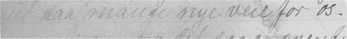ud saa mange nye veie for os.
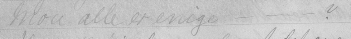Mon alle er enige - - - ?
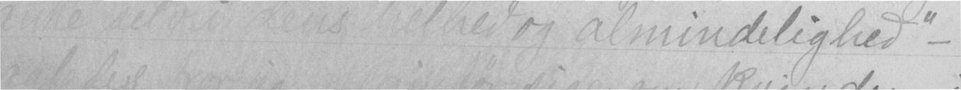tanke selv i dens helhed og almindelighed" -
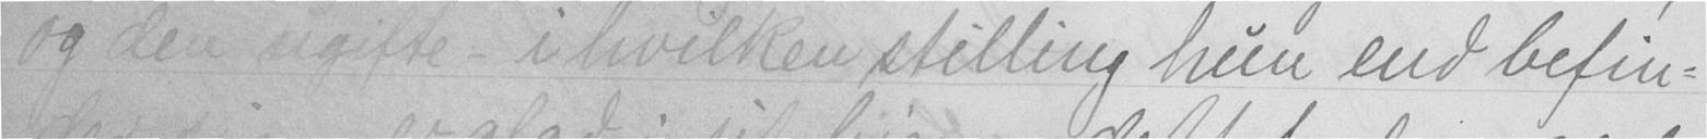og den ugifte, i hvilken stilling hun end befin-
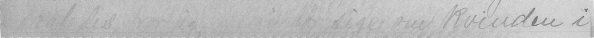saaledes tror jeg,   siger om kvinden i
